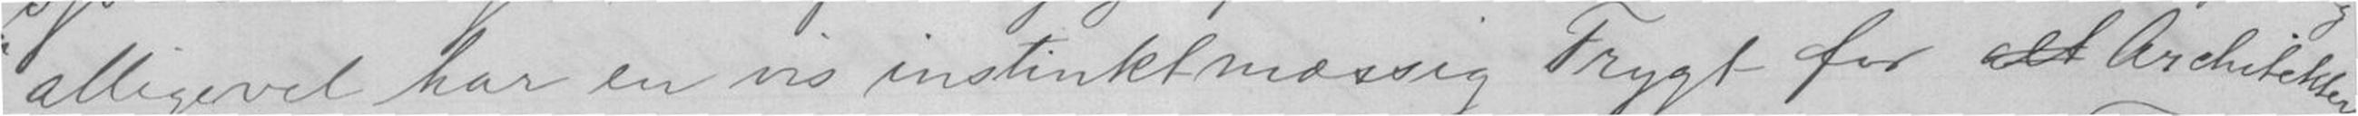alligevel har en vis instinktmæssig Frygt for alt Architekter
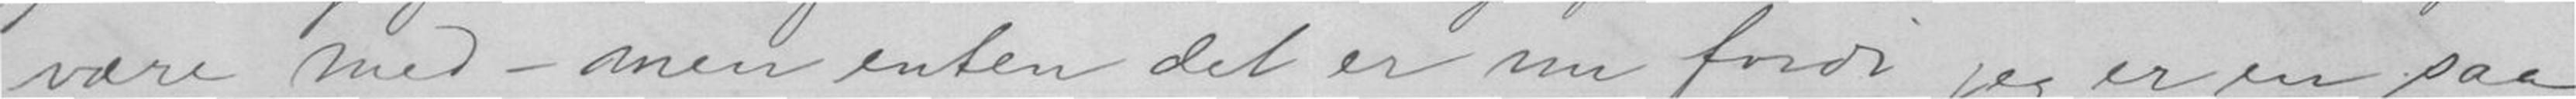være med - men enten det er nu fordi jeg er en saa
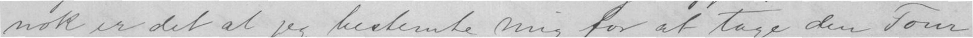nok er det at jeg bestemte mig for at tage den Tour
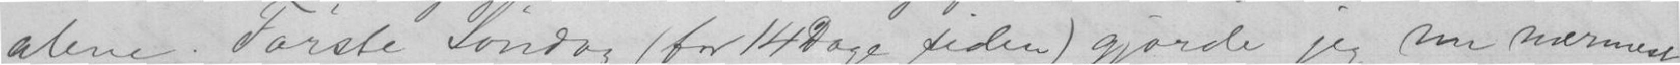alene. Første Søndag (for 14 Dage siden) gjorde jeg nu nærmest
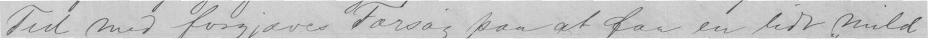Tid med forgjæves Forsøg paa at faa en lidt mild
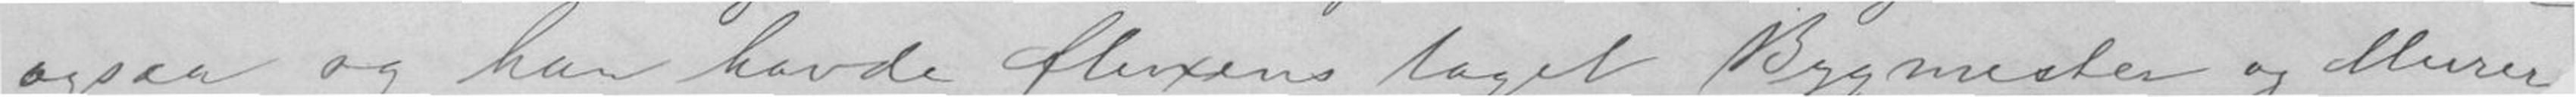ogsaa og han havde fluxens taget Bygmester og Murer
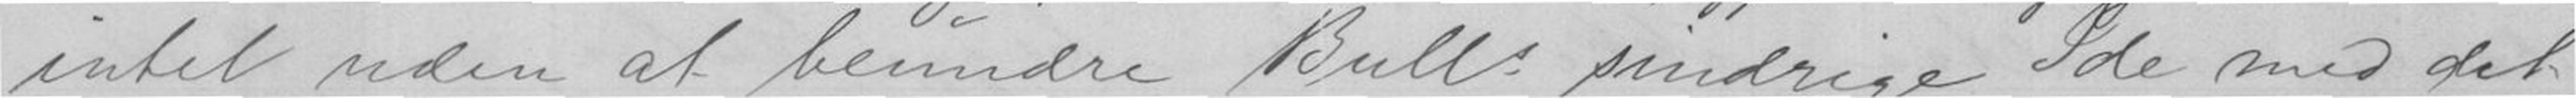intet uden at beundre Bulls sindrige Ide med det
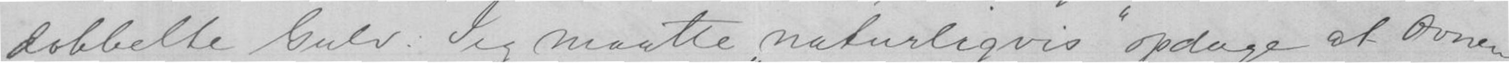dobbelte Gulv. Jeg maatte naturligvis opdage at Ovnen
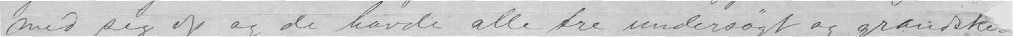med sig op og de havde alle tre undersøgt og granske
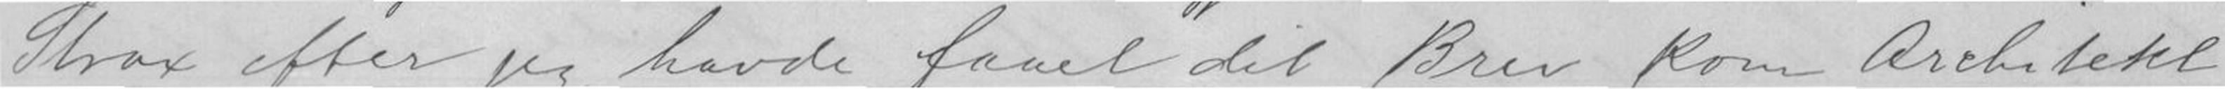Strax efter jeg havde faaet dit Brev, kom Architekt
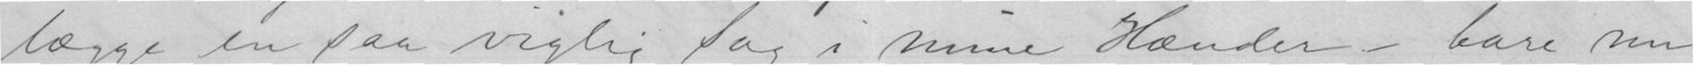lægge en saa vigtig Sag i mine Hænder - bare nu
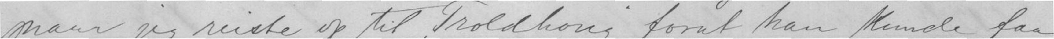naar jeg reiste op til Troldhoug forat han kunde faa
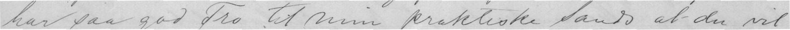har saa god Tro til min praktiske Sands at du vil
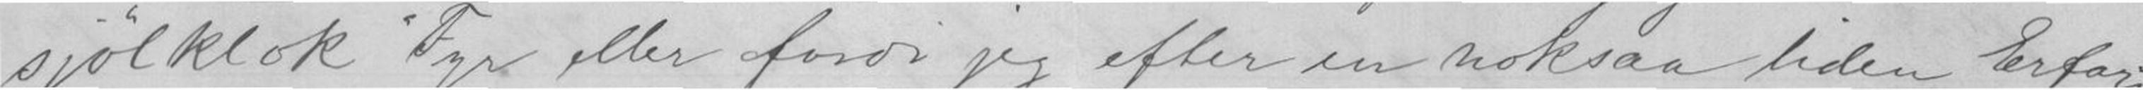sjølklok Fyr eller fordi jeg efter en noksaa liden Erfaring
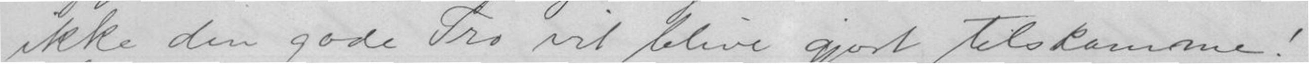ikke den gode Tro vil blive gjort tilskamme!
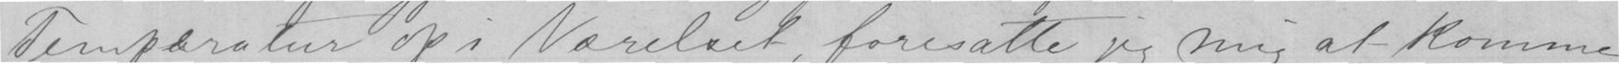Temperatur op i Værelse, foresatte jeg mig at komme
